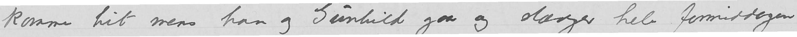komme hit mens han og Gunhild gaar og slænger hele formiddagen,
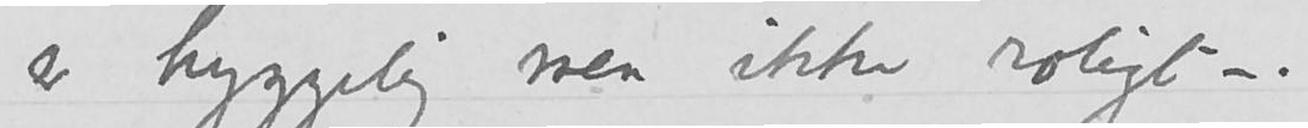er hyggelig men ikke roligt –.
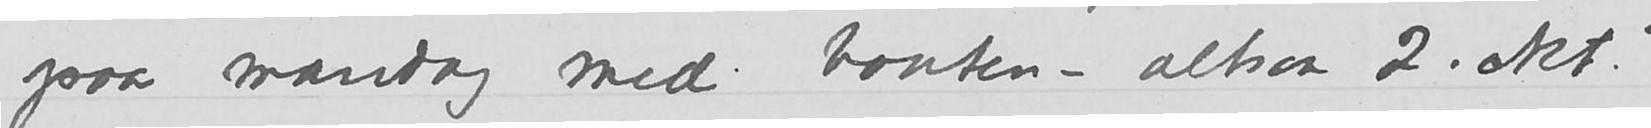paa mandag med baaten – altsaa 2. okt.?
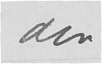din
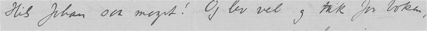Hils Johan saa moget! Og lev vel og tak for boken,
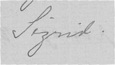Sigrid
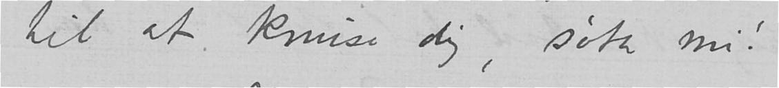til at knuse dig, søta mi!
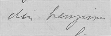din hengivne
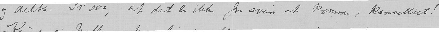og delta. Si saa at det er ikke for svin at komme i kancelliet!
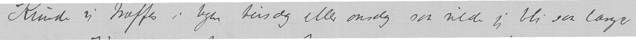Kunde vi træffes i byen tirsdag eller onsdag saa vilde jeg bli saa længe
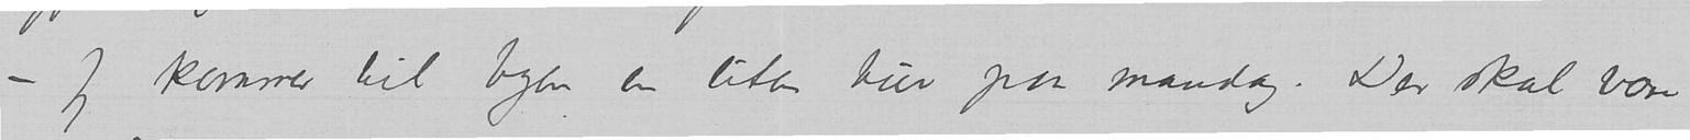– Jeg kommer til byen en liten tur paa mandag. Der skal være
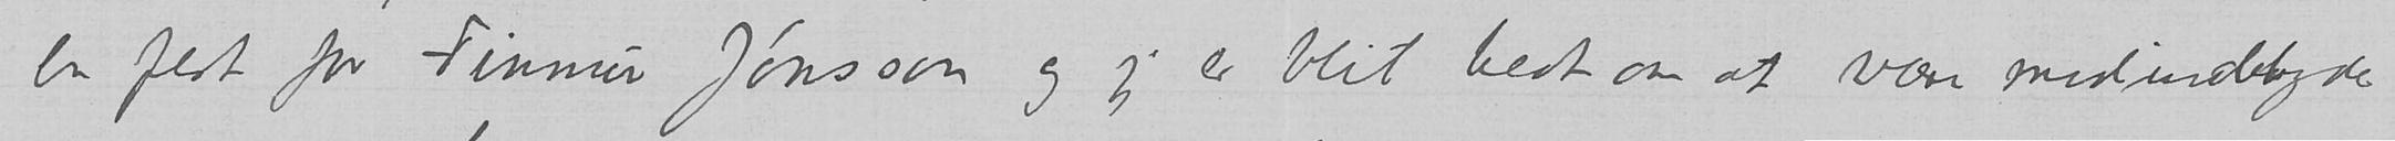en fest for Finnur Jónson og jeg er blit bedt om at være medindbyder
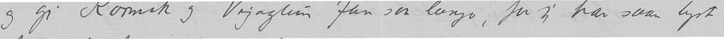og gi Kormak og Vigaglum fan saa længe, for jeg har saan lyst
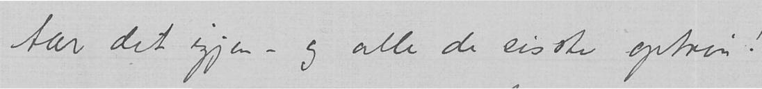tar det igjen – og alle de sisste optrin!
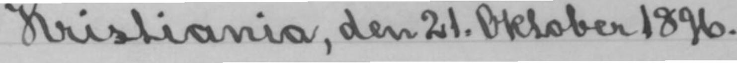Kristiania, den 21. Oktober 1896.
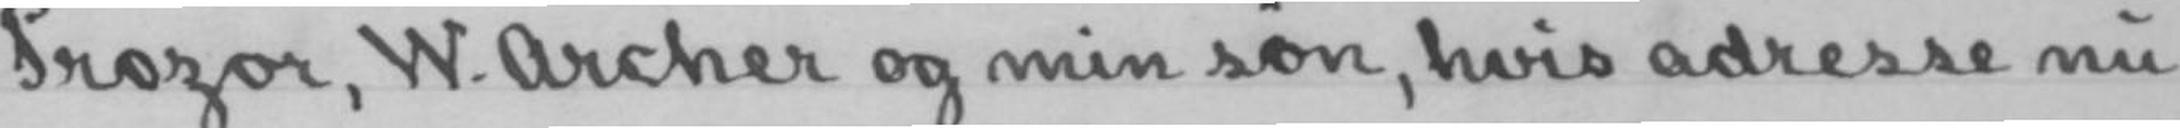Prozor, W. Archer og min søn, hvis adresse nu
